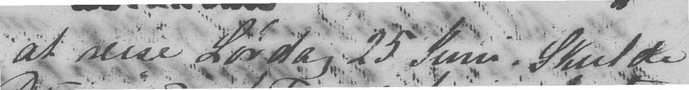at reise Lørdag 25 Juni. Skulde
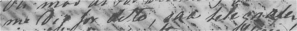me Dig for dette, saa telegrafer,
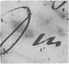Din
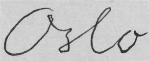Oslo
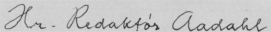Hr. Redaktør Aadahl
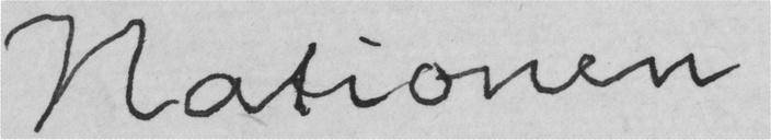Nationen
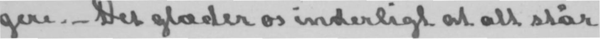gere.- Det glæder os inderligt at alt står
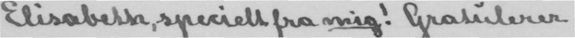Elisabeth, specielt fra mig! Gratulerer
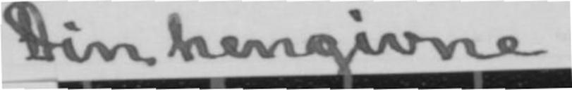Din hengivne
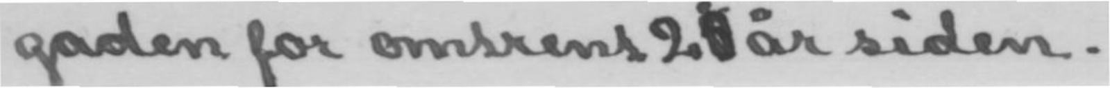gaden for omtrent 20 år siden.
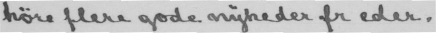høre flere gode nyheder fr eder.
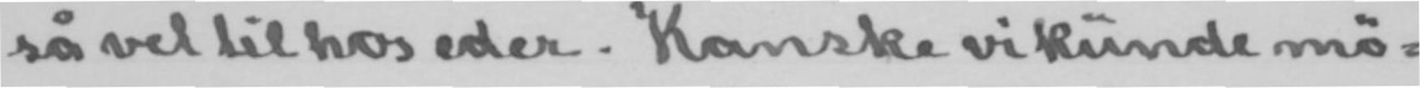så vel til hos eder. Kanske vi kunde mø-
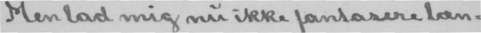Men lad mig nu ikke fantasere læn-
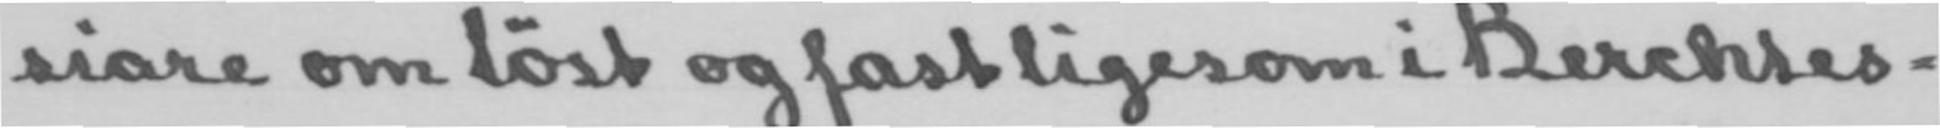siare om løst og fast ligesom i Berchtes-
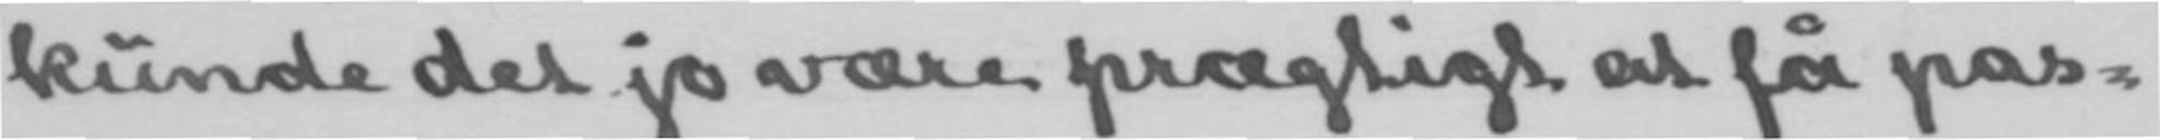kunde det jo være prægtigt at få pas-
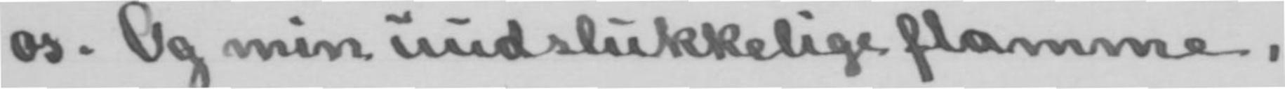os. Og min uudslukkelige flamme,
